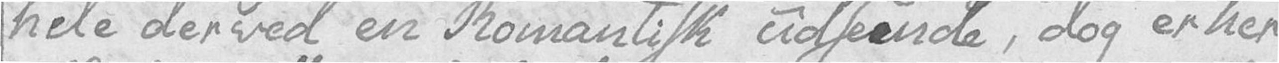hele derved en Romantisk udseende, dog er her
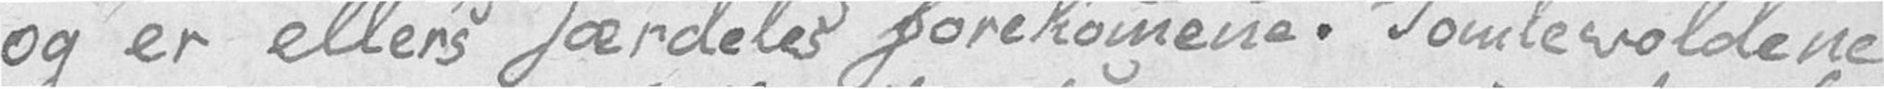og er ellers særdeles forekommene. Tomlevoldene
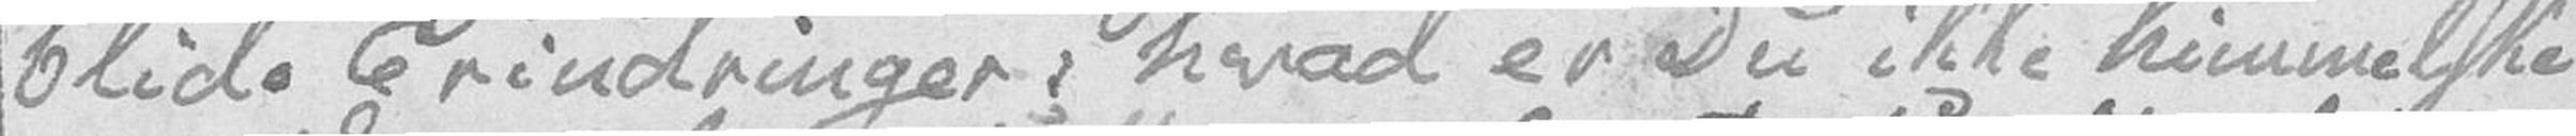blide Erindringer, hvad er Du ikke himmelske
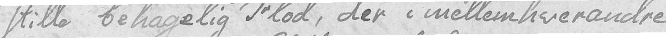stille behagelig Flod, der i mellem hverandre
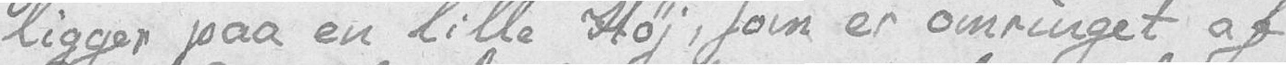ligge paa en lille Høj, som er omringet af
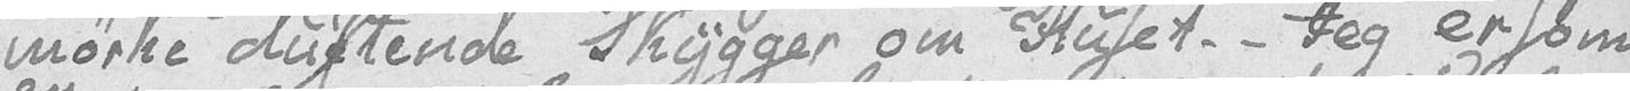mørke duftende Skygger om Huset. – Jeg er som
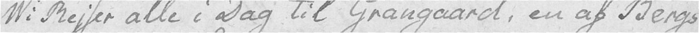Vi Rejser alle i Dag til Grangaard, en af Bergs
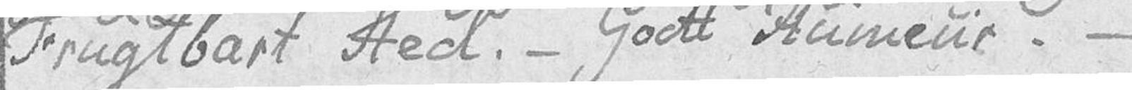Frugtbart Sted. – Godt Humeur. –
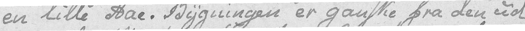en lille Aae. Bygningen er ganske fra den ud
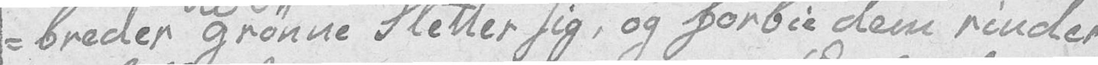-breder grønne Sletter sig, og forbie dem rinder
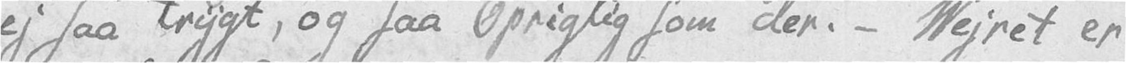ej saa trygt, og saa Oprigtig som der. – Vejret er
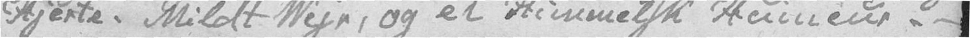Hjerte. Mildt Vejr, og et Himmelsk Humeur. –
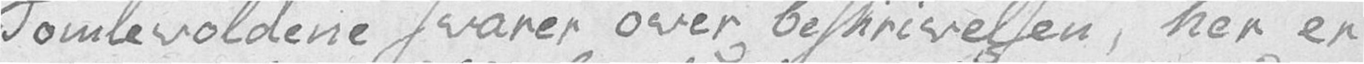Tomlevoldene svarer over beskrivelsen, her er
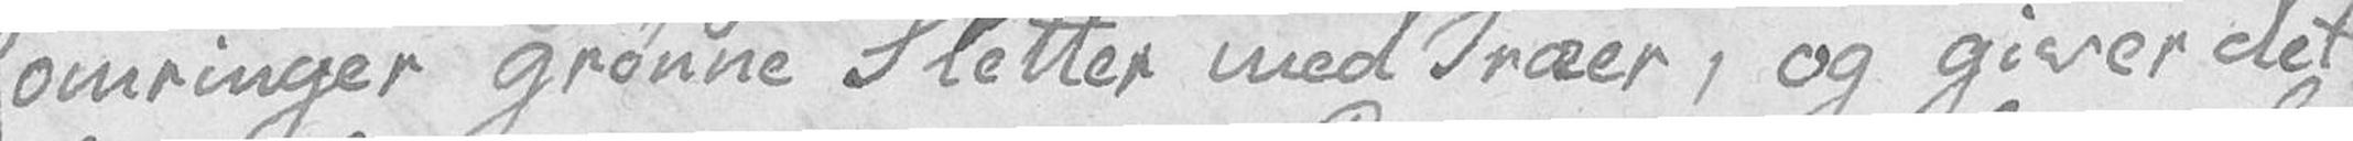omringer grønne Sletter med Træer, og giver det
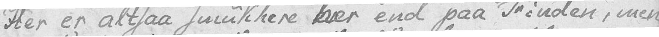Her er altsaa smukkere her end paa Finden, men
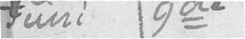Juni 9de
